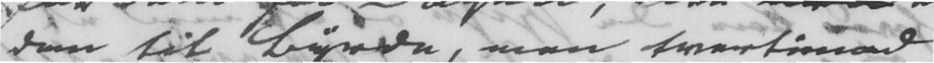dem til byrde, men tvertimod
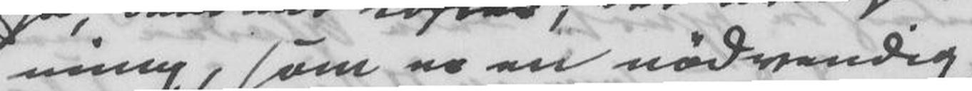ming, som er nu nødvendig
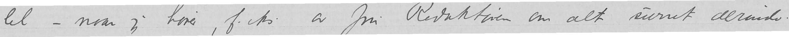lel – naar jeg hører, f.eks. av fru Redaktøren om alt surret derinde.
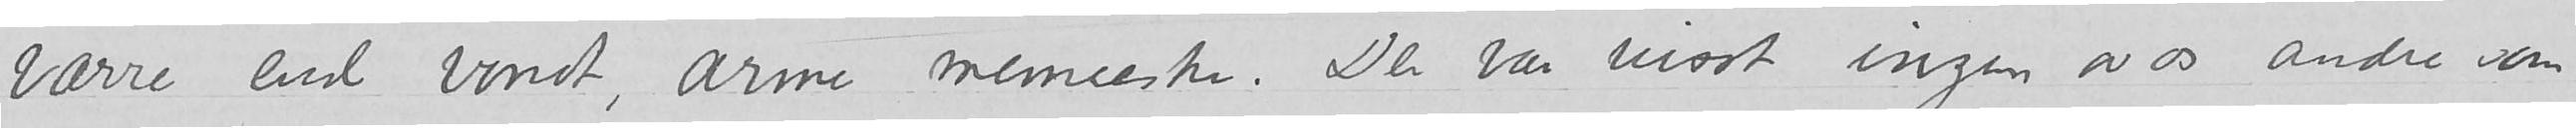værre endt vondt, arme menneske. Der var visst ingen av os andre som
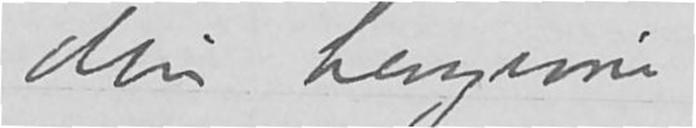din hengivne
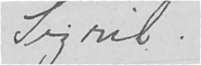Sigrid.
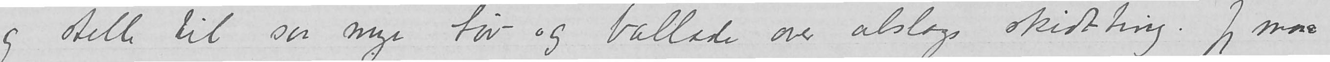og stelle til saa mye liv og ballader over alslags skidtting. Jeg maa
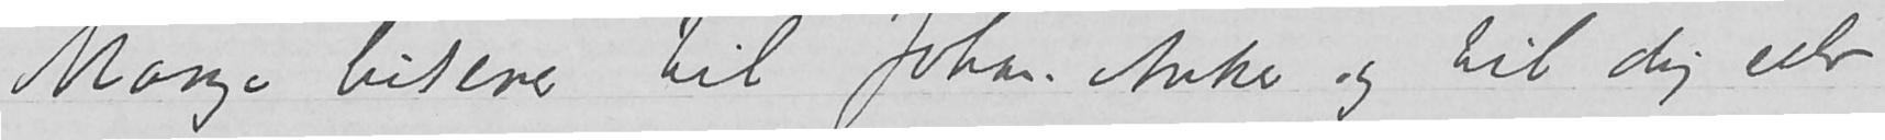Mange hilsener til Johan Anker og til dig selv
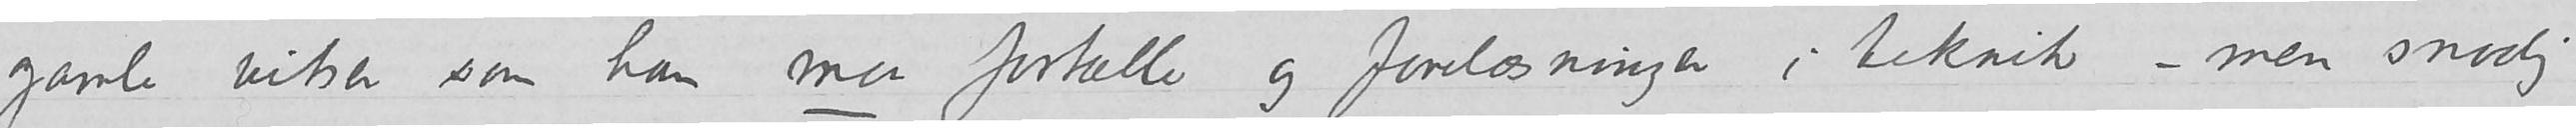gamle vitser som han maa fortælle og forelæsninger i teknik – men snodig
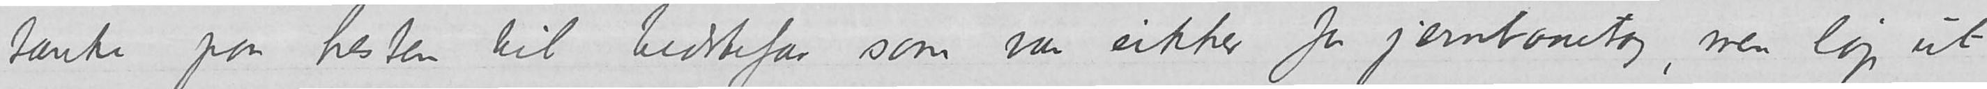tænke paa hesten til bestefar som var sikker for jernbanetog, men løp ut
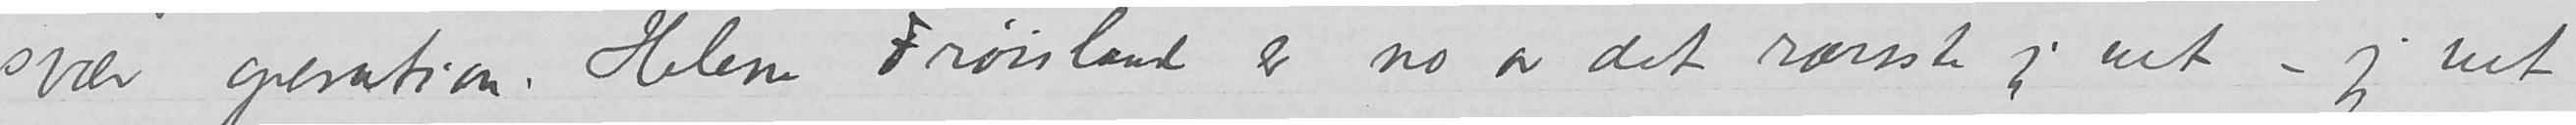svær operation. Helene Frøisland er no av det særeste jeg vet
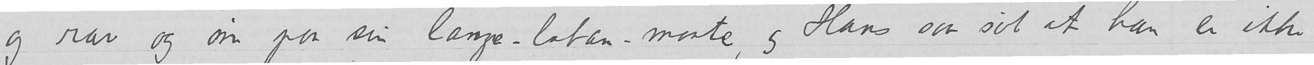og rar og øm paa sin lange-laban-maateorte, og Hans saa søt at han er ikke
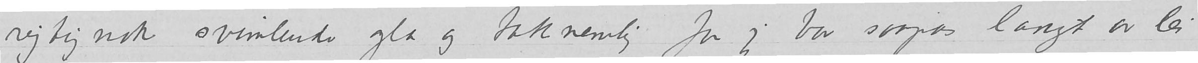riktignok svimlende gla og taknemlig for jeg bor saapas langt av lei
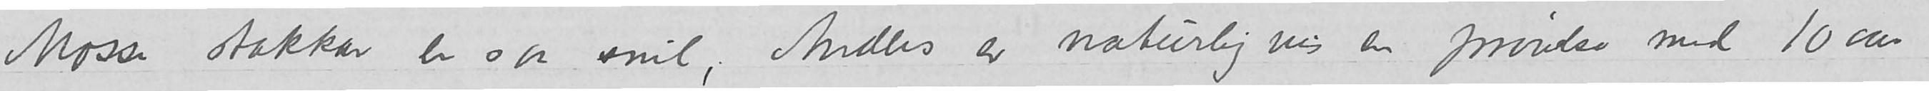Mosse stakkar er saa snil; Anders er naturligvis en prøvelse med 10 aar
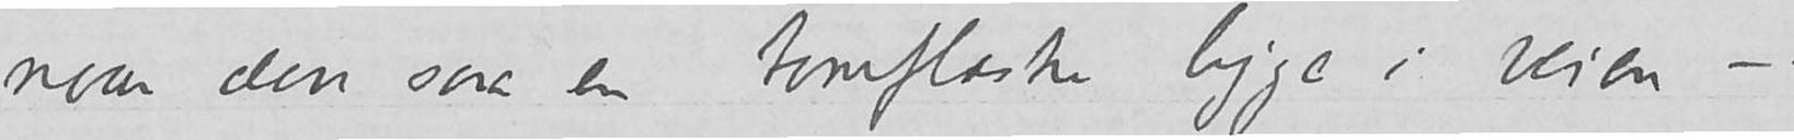naar den saa en tomflaske ligge i veien –.
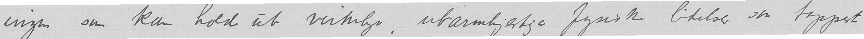– jeg vet ingen som kan holde ut virkelige, ubarmhjertige fysiske lidelser saa tappert
